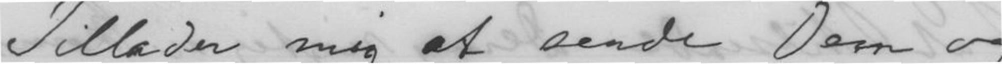Tillader mig at sende Dem og
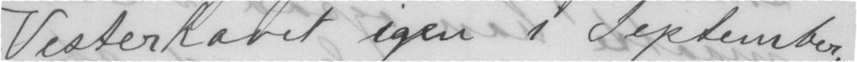Vesterhavet igen i September,
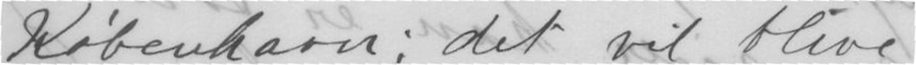København; det vil blive
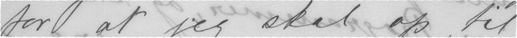for at jeg skal op til
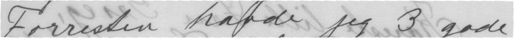Forresten havde jeg 3 gode
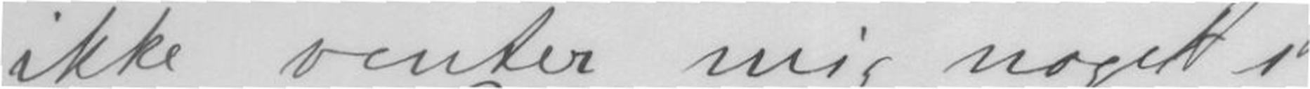ikke venter mig noget i
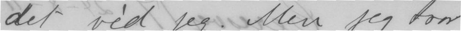det ved jeg. Men jeg tror
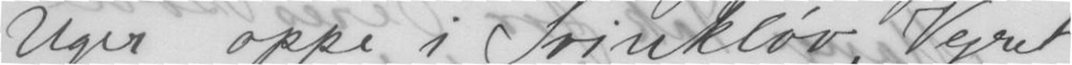Uger oppe i Svinkløv, Vejret
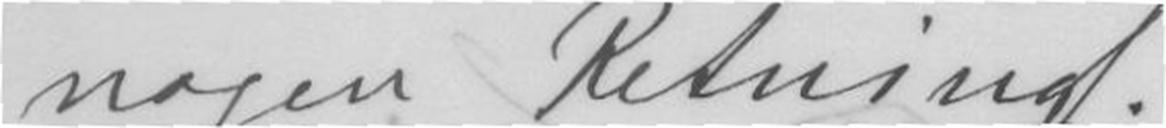nogen Retning.
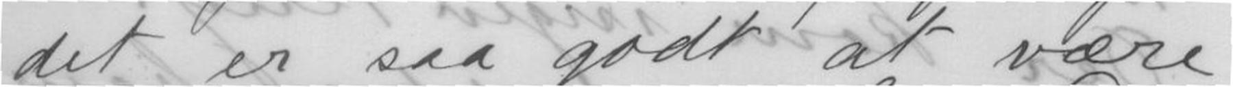det er saa godt at være
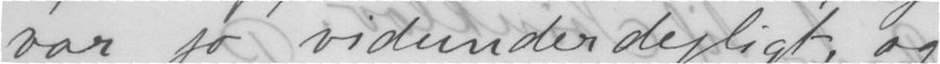var jo vidunderdejligt, og
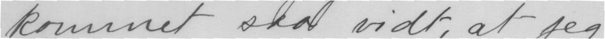kommet saa vidt, at jeg
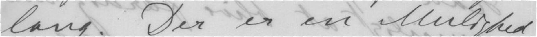lang. Der er en Mulighed
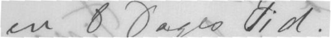en 8 Dagers Tid.
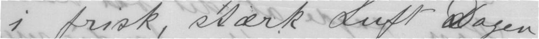i frisk, stærk Luft Dagen
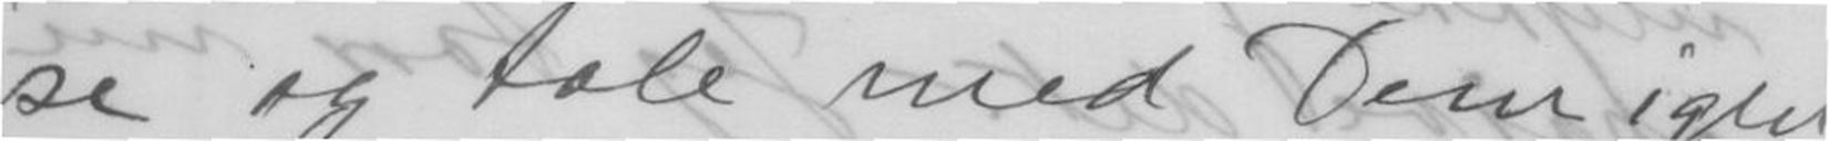se og tale med Dem igen.
